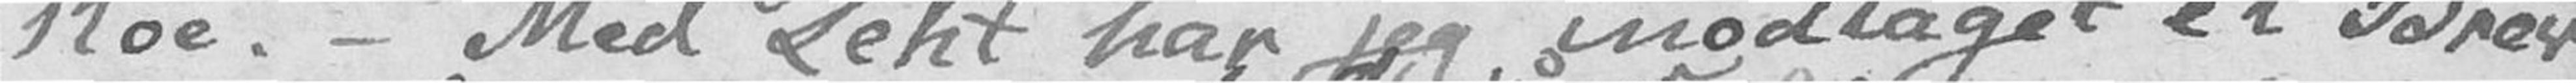Roe. – Med Leht har jeg modtaget et Brev
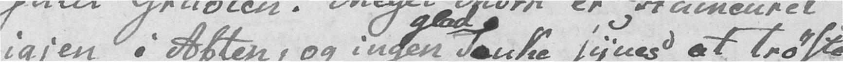igjen i Aften, og ingen glad Tanke synes at trøste
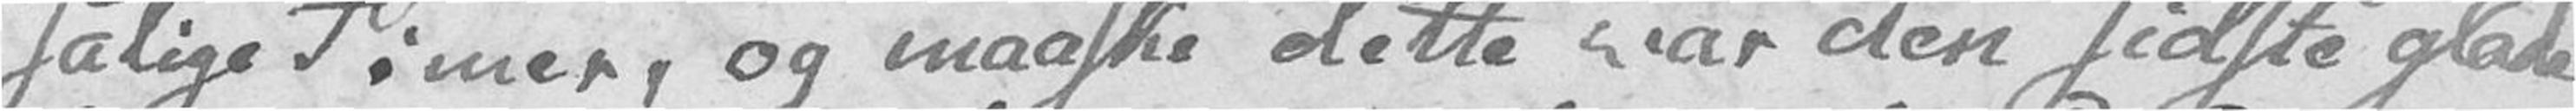salige Timer, og maaske dette var den sidste glade
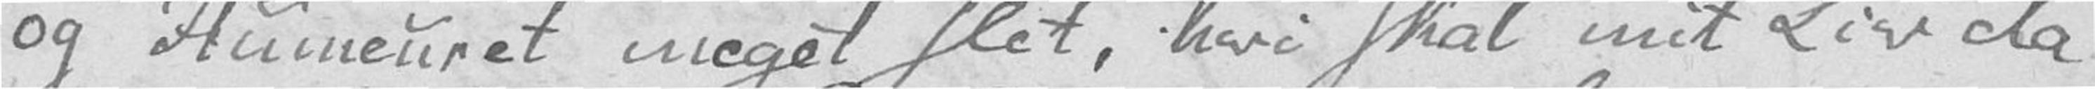og Humeuret meget slet, hvi skal mit Liv da
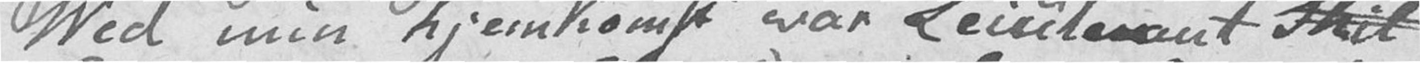Ved min hjemkomst var Leiutenant Shil
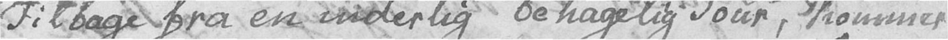Tilbage fra en inderlig behagelig Tour, kommer
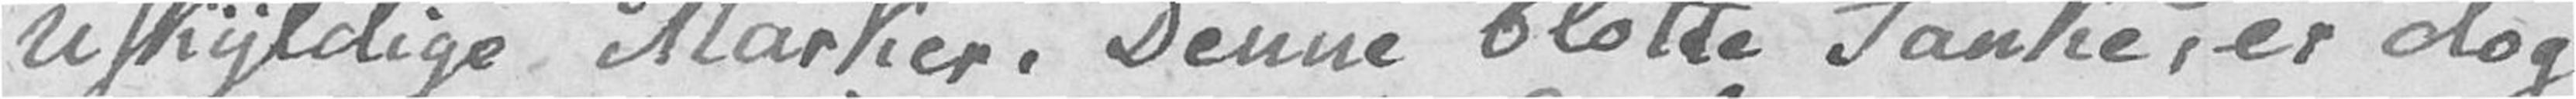uskyldige Marker. Denne blotte Tanke, er dog
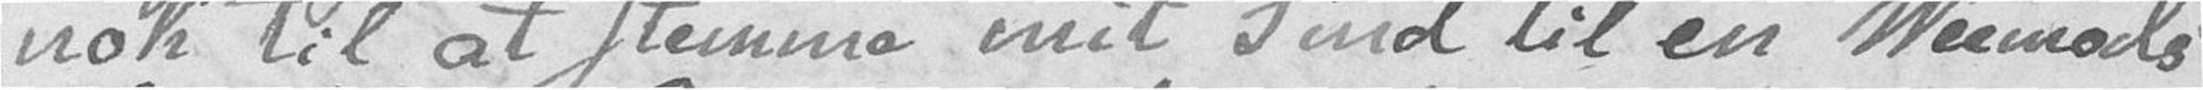nok til at stemme mit Sind til en Veemods
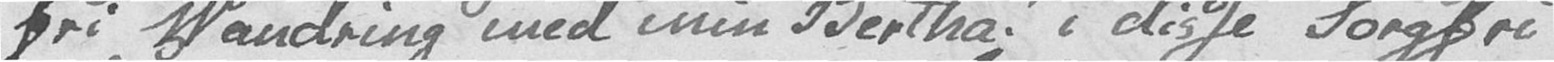fri Vandring med min Bertha! i disse Sorgfri
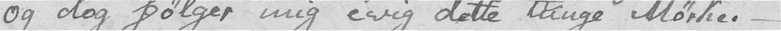og dog følger mig evig dette tunge Mørke. –
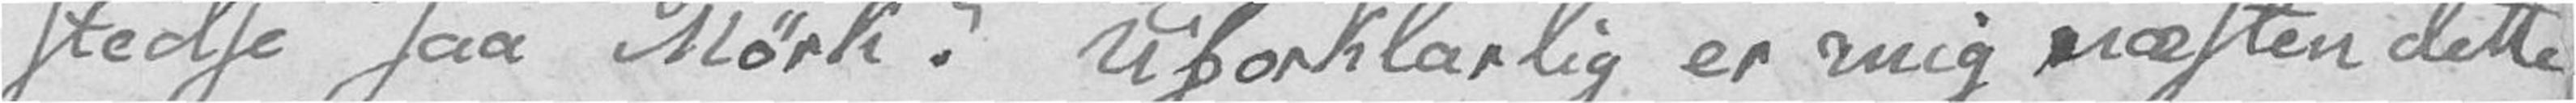stedse saa Mørk? Uforklarlig er mig næsten dette
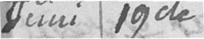Juni 19de
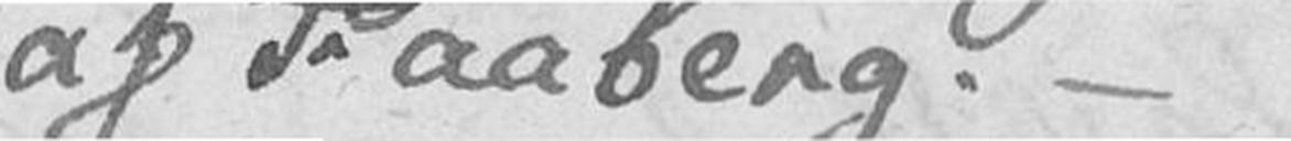af Faaberg. –
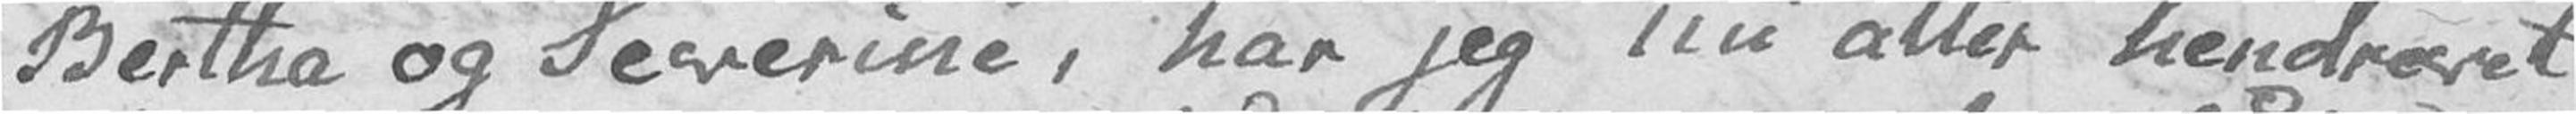Bertha og Severine! har jeg nu atter hendrevet
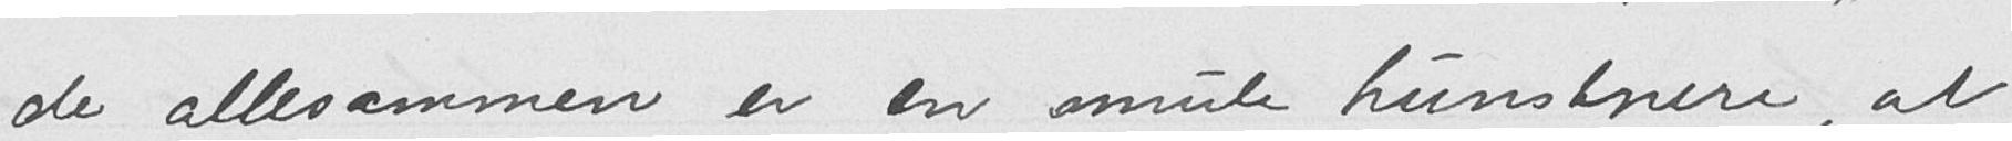de allesammen er en smule kunstnere, at
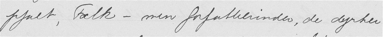pjalt, Falk - men forfatterinder, de dyrker
